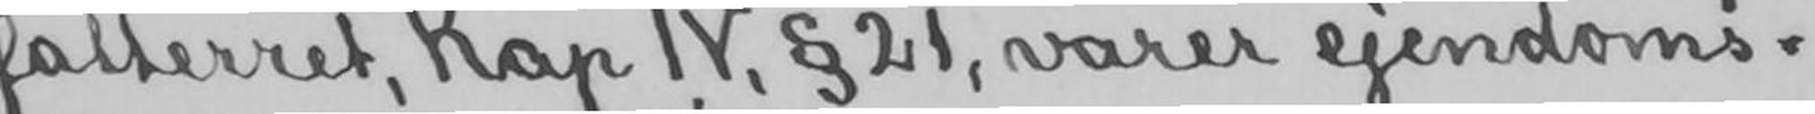fatterret, Kap IV, § 21, varer ejendoms-
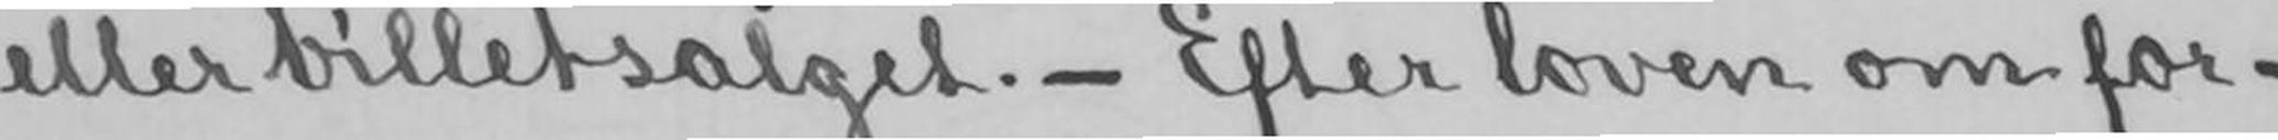eller billetsalget.- Efter loven om for-
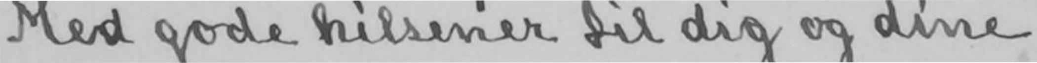Med gode hilsener til dig og dine
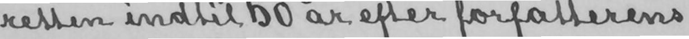retten indtil 50 år efter forfatterens
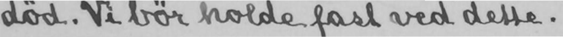død. Vi bør holde fast ved dette.
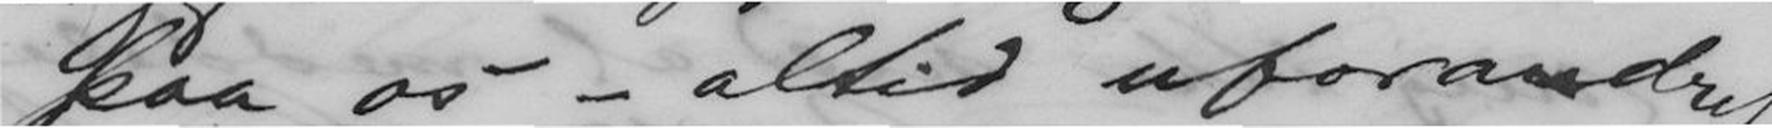paa os - altid uforandret!
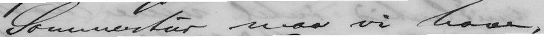Sommertur maa vi have,
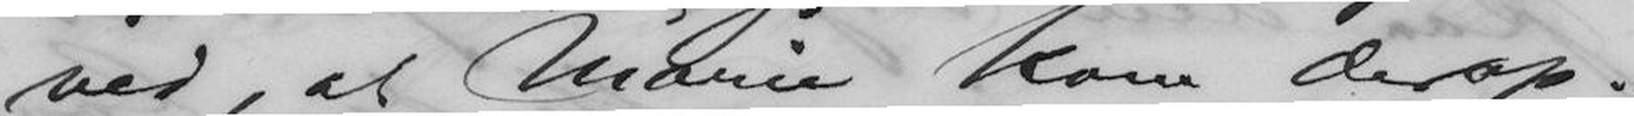ved, at Marie kom derop.
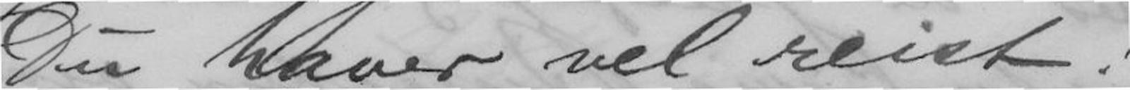"Du haver vel reist!"
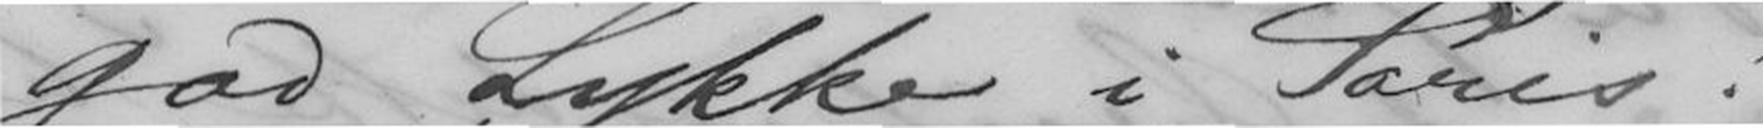god Lykke i Paris!
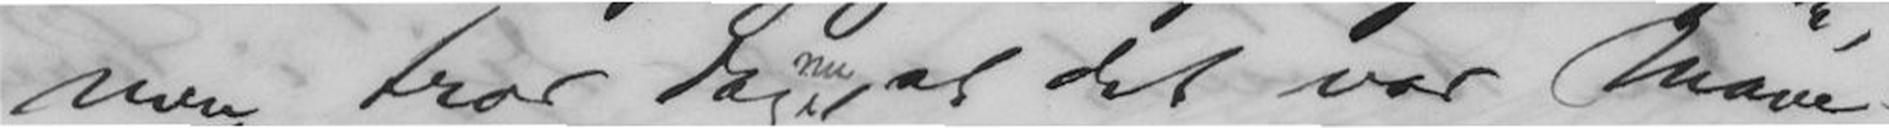men tror dog nu, at det var Mave-
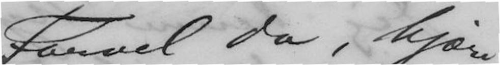Farvel da, kjære
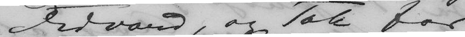Edvard, og Tak for
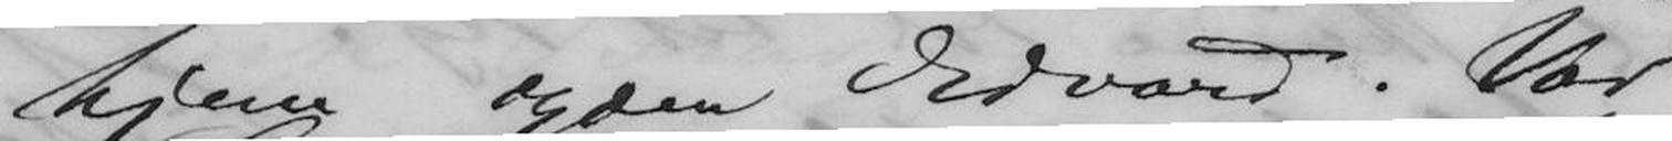hjem igjen Edvard. Vor
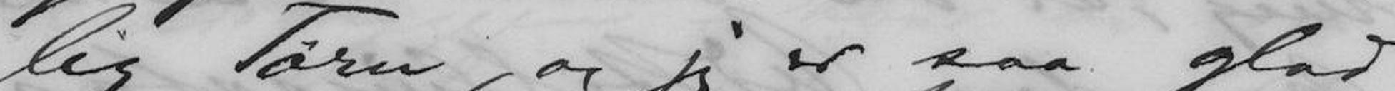lig Tørn, og jeg er saa glad
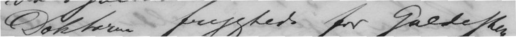Doktoren frygtede for Galdesten,
